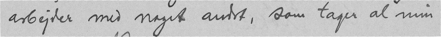arbejder med noget andet, som tager al min
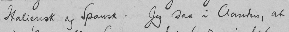Italiensk og Spansk. Jeg saa i Aanden, at
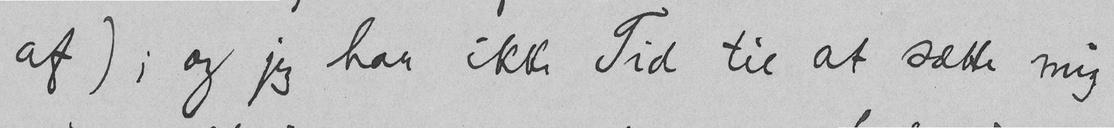af); og jeg har ikke Tid til at sætte mig
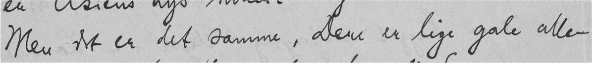Men det er det samme, Dere er lige gale alle
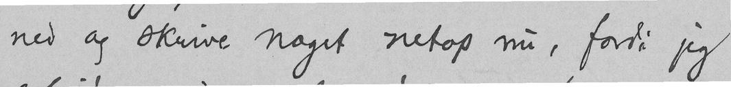ned og skrive noget netop nu, fordi jeg
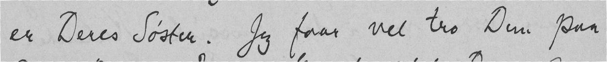er Deres Søster. Jeg faar vel tro Dem paa
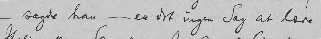- sagde han - er det ingen Sag at lære
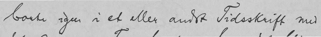borte igen i et eller andet Tidsskrift med
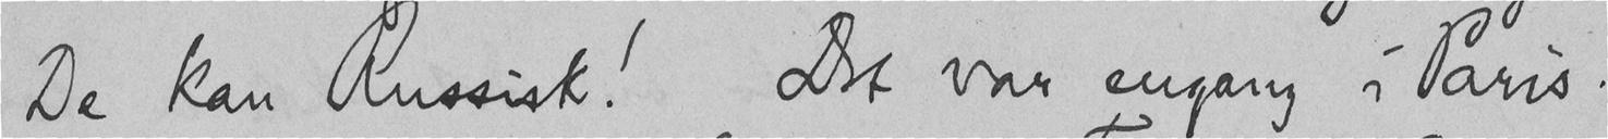De kan Russisk! Det var engang i Paris
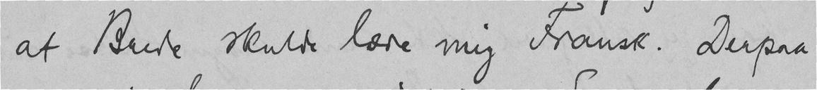at Brede skulde lære mig Fransk. Derpaa
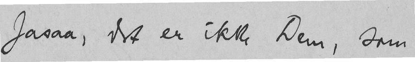Jasaa, det er ikke Dem, som
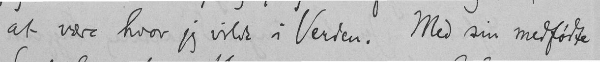at være hvor jeg vilde i Verden. Med sin medfødte
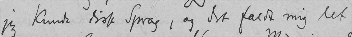jeg kunde disse Sprog, og det faldt mig let
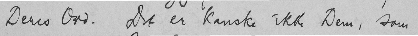Deres Ord. Det er kanske ikke Dem, som
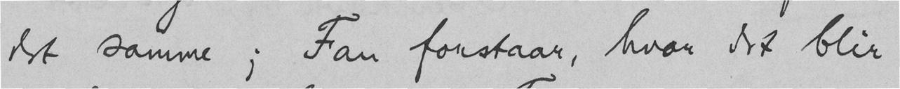det samme; Fan forstaar, hvor det blir
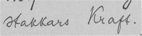stakkars Kraft.
